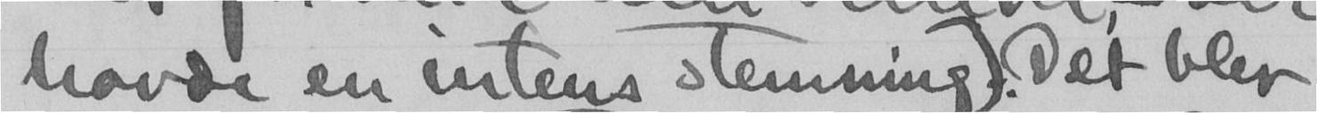havde en intens stemning). Det blev
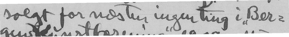solgt for næsten ingenting i "Ber-
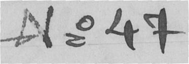No 47
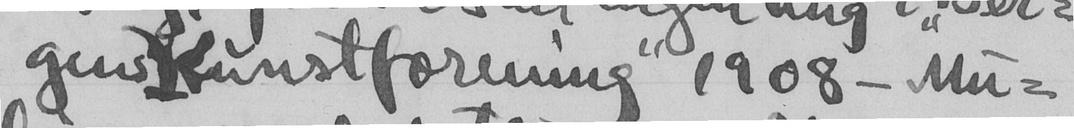gens Kunstforening" 1908 - Mu-
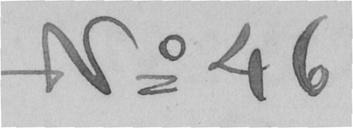No 46
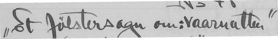"Et Jølstersagn om: Vaarnatten"
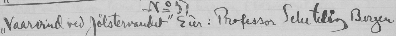"Vaarvind ved Jølstervandet" Eier: Professor Schetelig Bergen
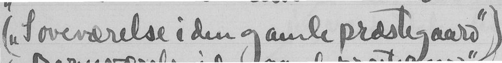("Soveværelse i den gamle præstegaard")
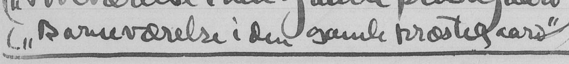("Barneværelse i den gamle præstegaard")
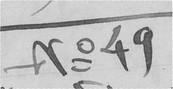No 49
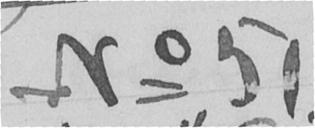No 51
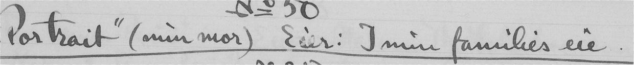"Portrait" (min mor) Eier: I min families eie.
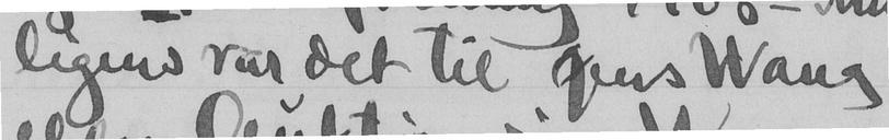ligens var det til Jens Wang
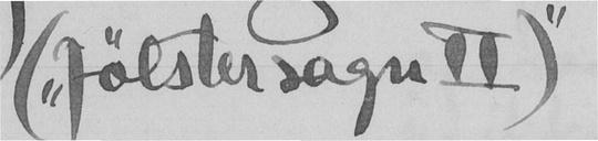("Jølstersagn II")
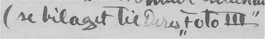(se bilaget til Deres "Foto III"
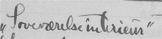"Soveværelseinterieur"
